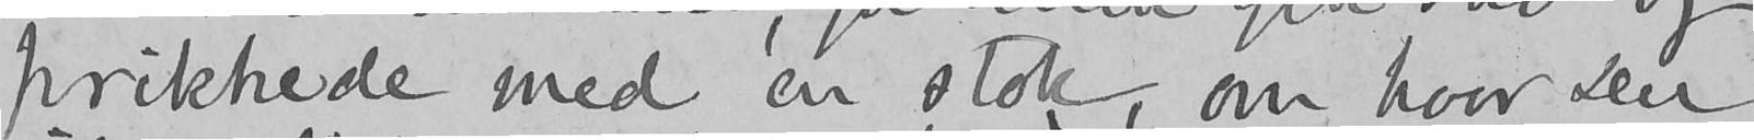prikkede med en stok, om hvor Du
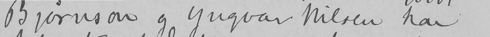Bjørnson og Yngvar Nilsen har
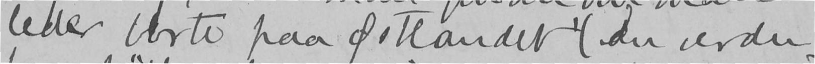leder borte paa Østlandet (du verden
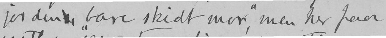jordene, "bare skidt mor", men her paa
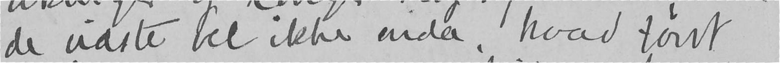de vidste vel ikke enda, hvad først
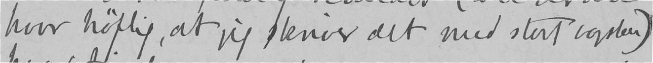hvor høflig, at jeg skriver det med stort bogstav)
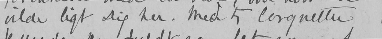vilde ligt Dig her. Med 5 lorgnetter
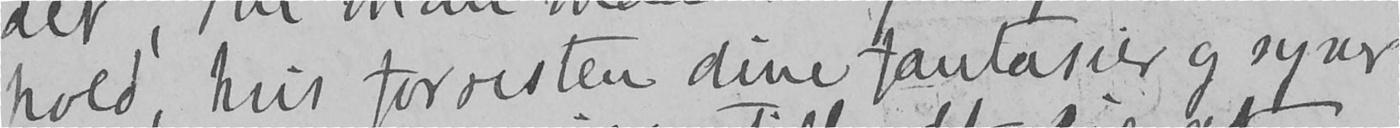hold, hvis forresten dine fantasier og syner
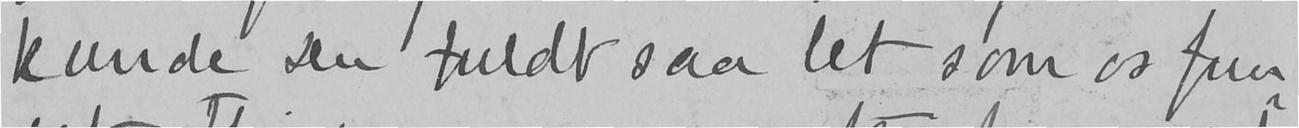kunde Du fuldt saa let som os fun-
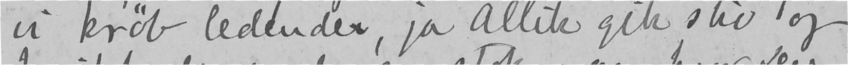vi krøb ledender, ja Allik gik stiv og
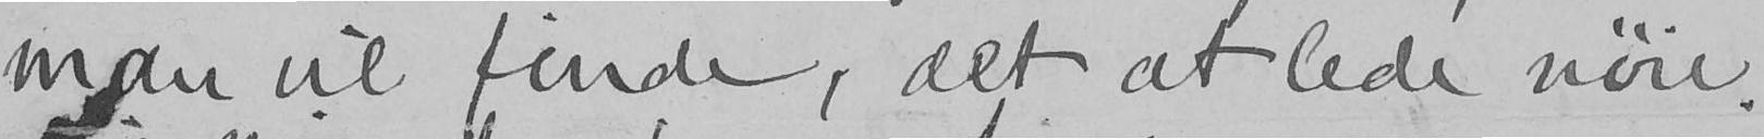man vil finde, det at lede nøie.
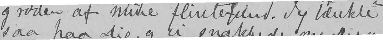grøden af mine flintefund. Jeg tænkte
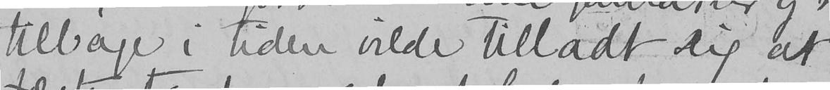tilbage i tiden vilde tilladt Dig at
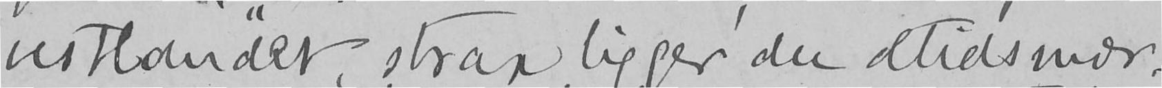vestlandet, strax ligger der oltidsmær-
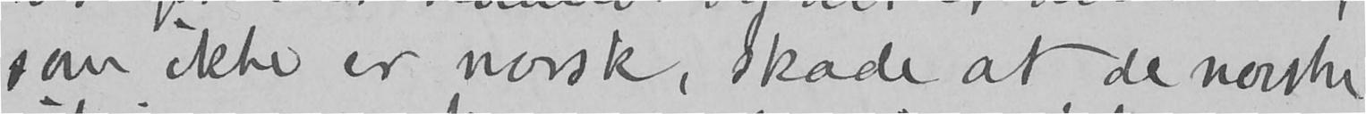som ikke er norsk, skade at de norske
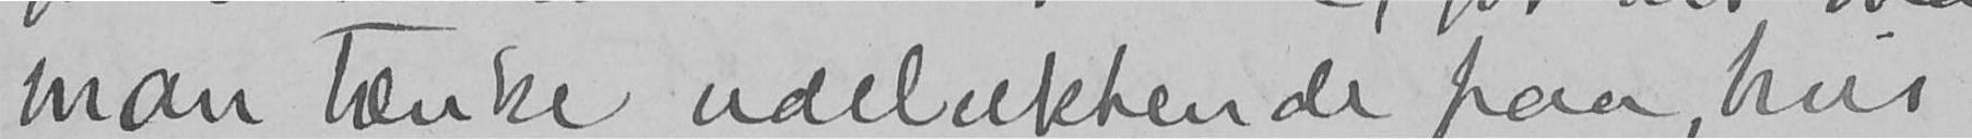man tænke udelukkende paa, hvis
